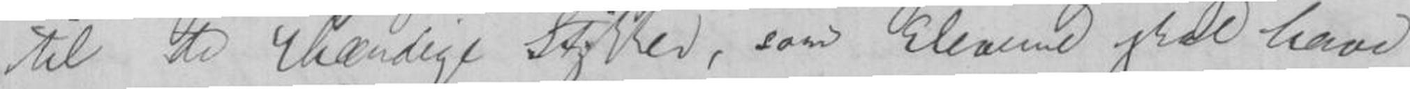til de 4hændige Stykker, som Eleverne skal have
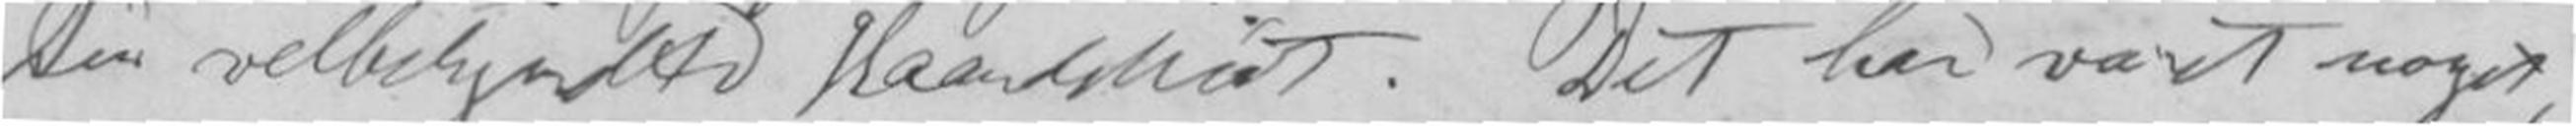Din velbekjendte Haandskrift. Det har været noget,
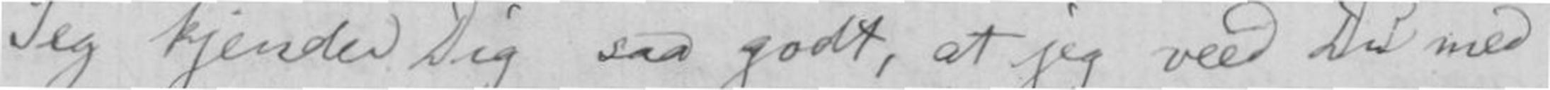Jeg kjender Dig saa godt, at jeg veed Du med
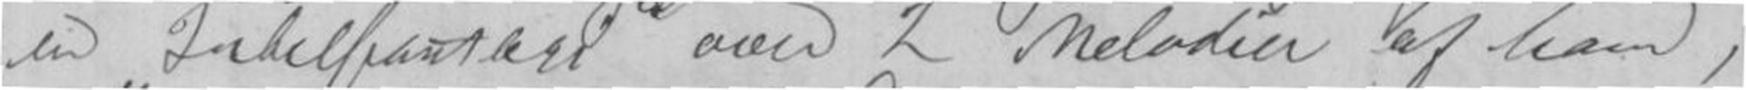en Jubelfantasi over 2 Melodier af ham;
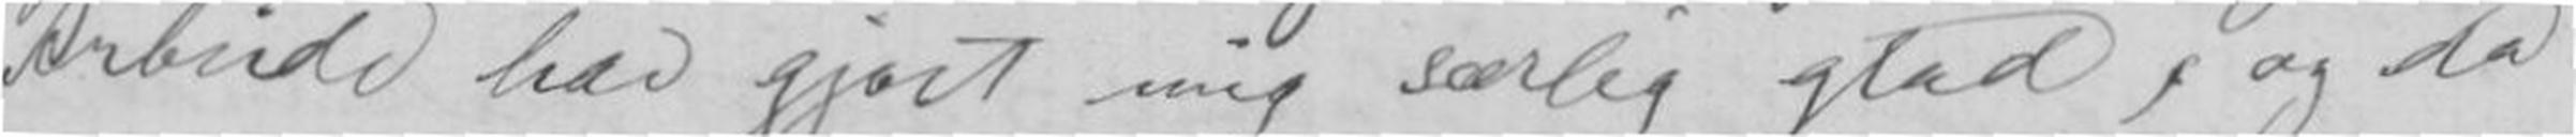Arbeide har gjort mig særlig glad; og da
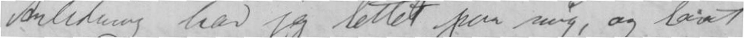Anledning har jeg lettet paa mig, og lavet
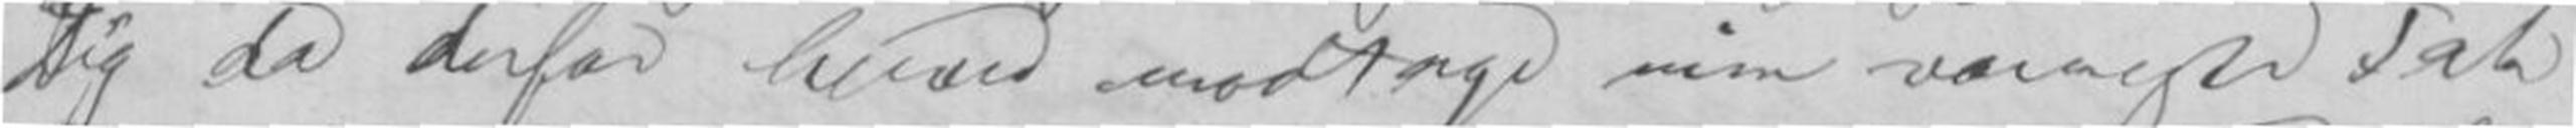Dig da derfor herved modtage min varmeste Tak
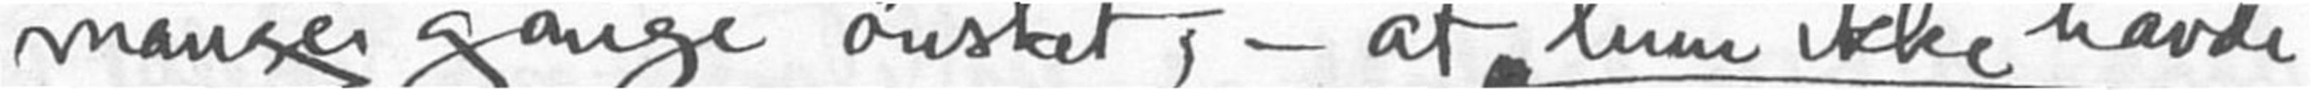mange gange ønsket, - at hun ikke havde
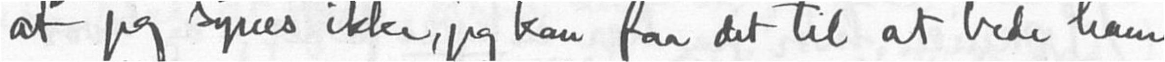at jeg synes ikke, jeg kan faa det til at bede ham
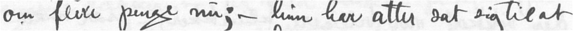om flere penge nu ; - hun har atter sat sig til at
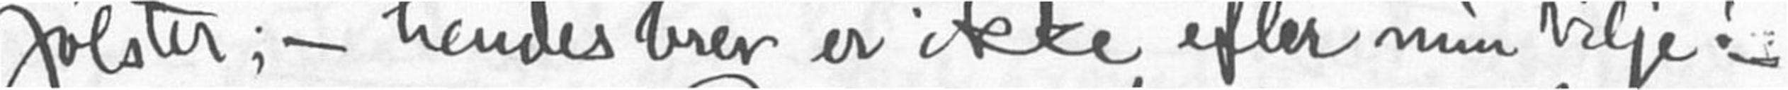Jølster; - hendes brev er ikke efter min vilje !
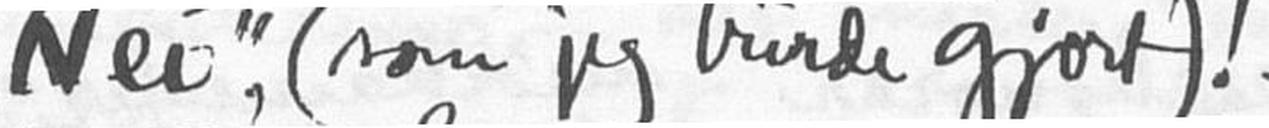"Nei", (som jeg burde gjort)!
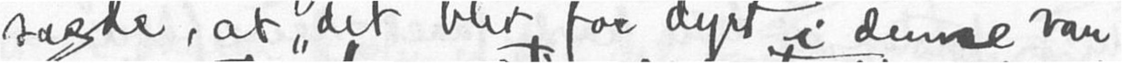sagde, at "det blir for dyrt" i denne van-
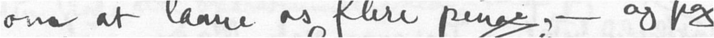om at laane os flere penge, - og jeg
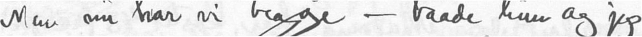Men nu har vi begge - baade hun og jeg -
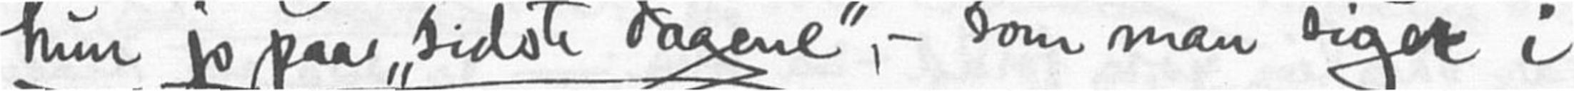hun jo paa "sidste dagene", - som man siger i
